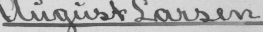August Larsen,
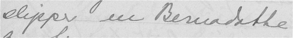slipper en Bernadotte
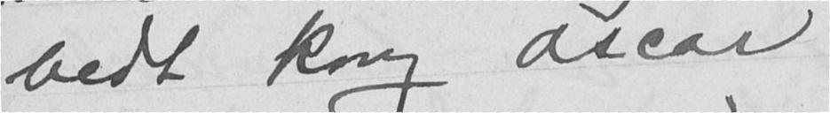bedt kong Oscar
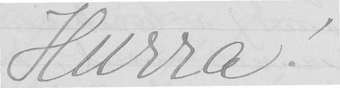Hurra!
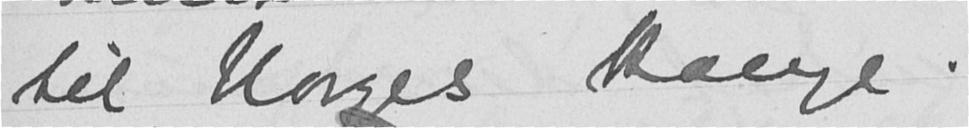til Norges konge.
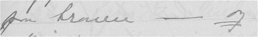paa tronen - og
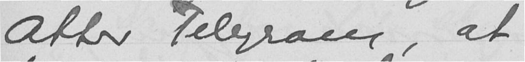Atter Telegram, at
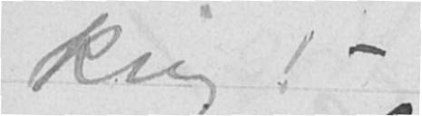krig! -
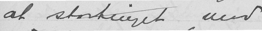at stortinget med
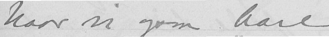Naar vi ogsaa bare
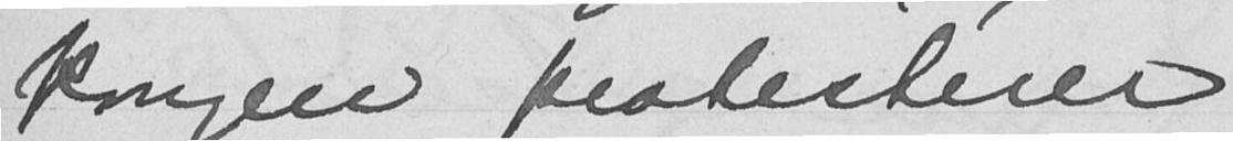kongen protesterer
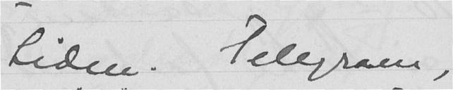Siden. Telegram,
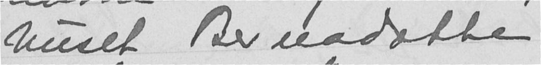huset Bernadotte
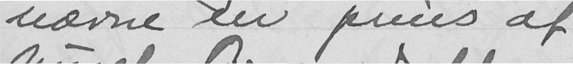nævne en prins af
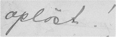opløst!
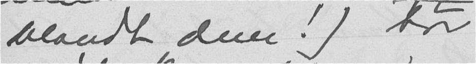blandt dem!) har
